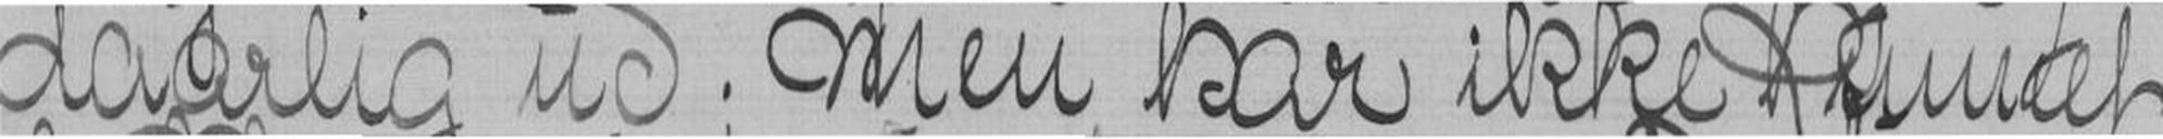daarlig ud; men har ikke kundet
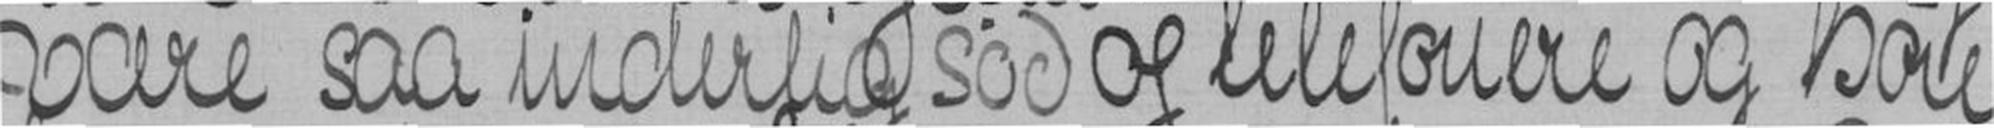være saa inderlig sød og telefonere og høre
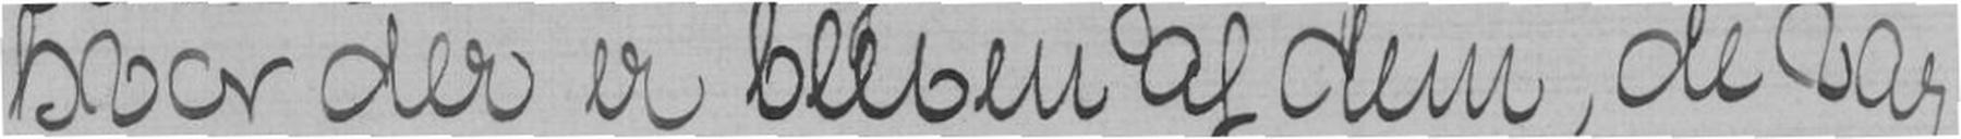hvor der er bleven af dem, de var
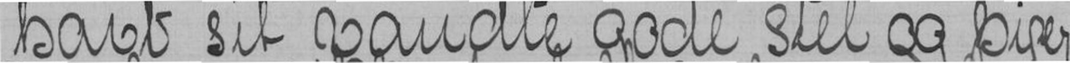havt sit vandte gode stel og piger
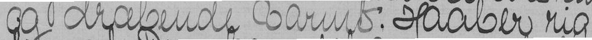og dræbende varmt. Haaber rig
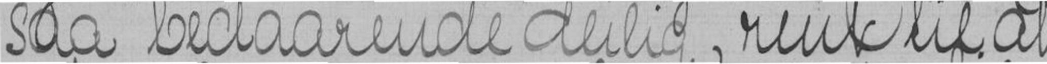saa bedaarende deilig, rent til at
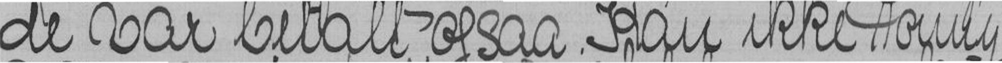de var betalt ogsaa. Kan ikke Tonny
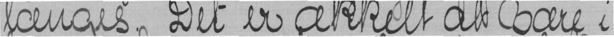længes. Det er ækkelt at være i
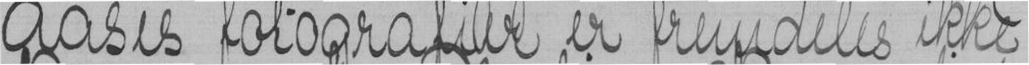Aases fotografier er fremdeles ikke
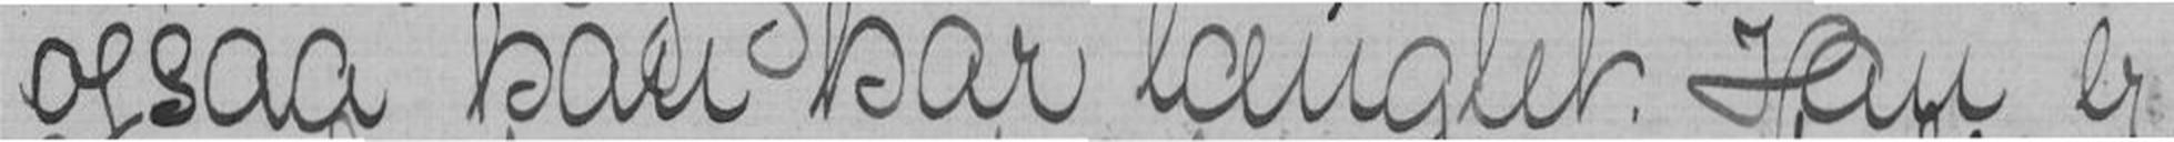ogsaa han har længtet. Han er
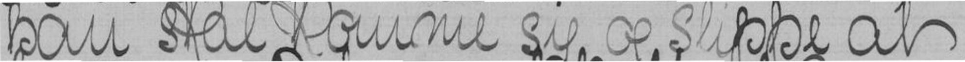han skal komme sig og slippe at
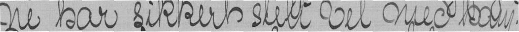ne har sikkert stelt vel med ham.
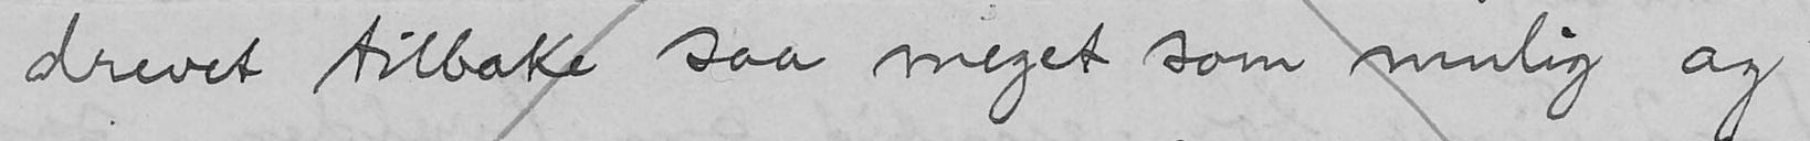drevet tilbake saa meget som mulig og
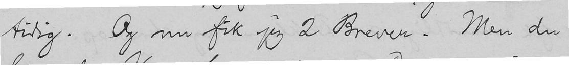tidig. Og nu fik jeg 2 Brever. Men du
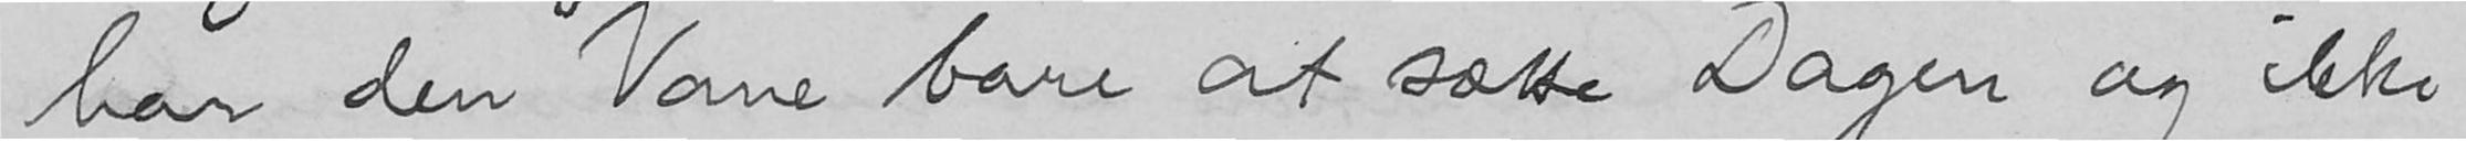har den Vane bare at sætte Dagen og ikke
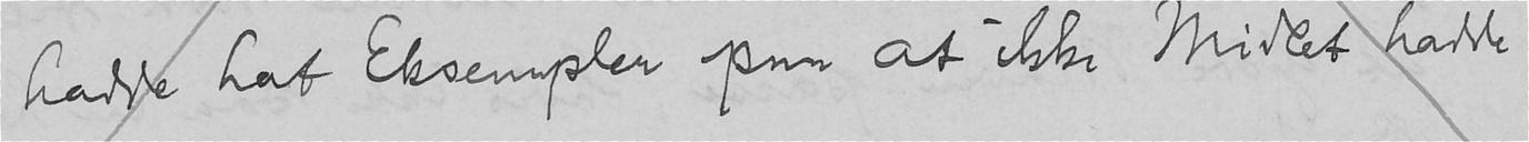hadde hat Eksempler paa at ikke Midlet hadde
